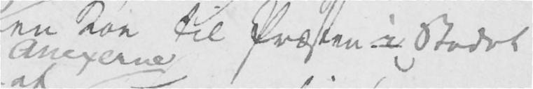en Koe til Præsten i Stedet
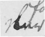der
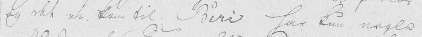og det vi kom til. Biri har kun nogle
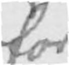for
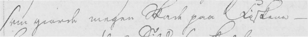som giorde megen Skade paa Fiskene -
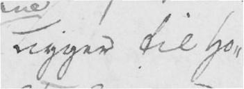ligger til Ho-
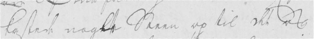kastede nogle Steen op til det og
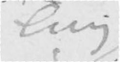lig
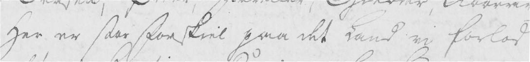Her er stor Forskiel paa det Land vi forlod
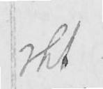det
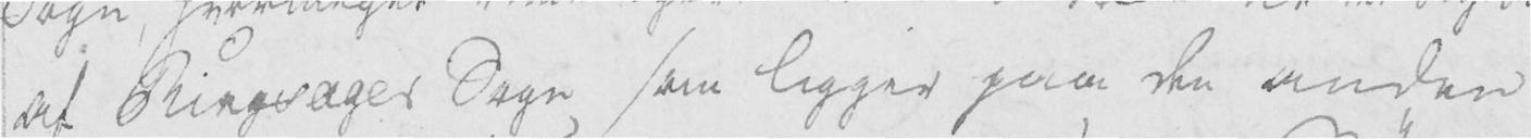af Ringsager Sogn som ligger paa den anden
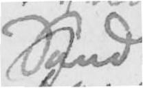Sand
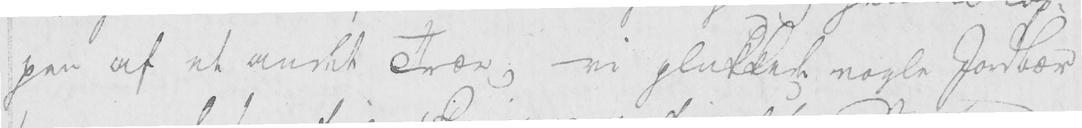pen af et andet Træe; vi plukkede nogle Jordbær
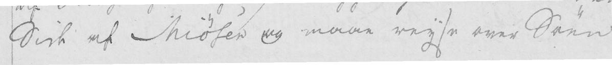Side af Miøsen og maae reyse over Søen
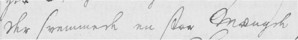der svemmede en stor Mængde
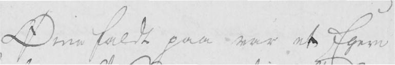Øine faldt paa var et Egorn
